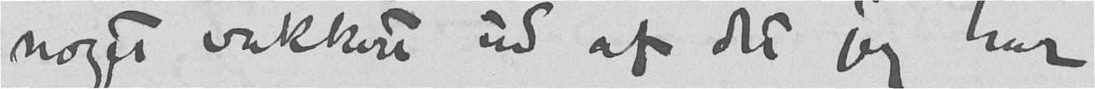noget vakkert ud af det jeg har
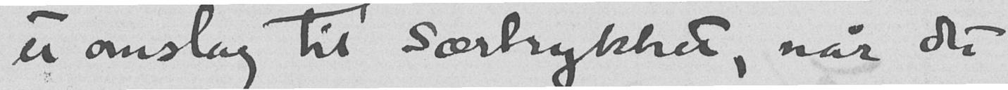et omslag til særtrykket, når det
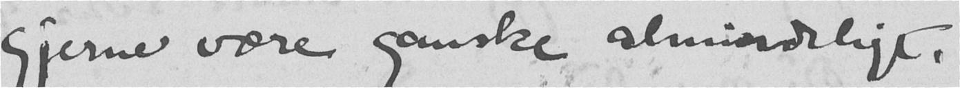gjerne være ganske almindligt.
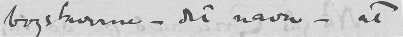bogstaverne - det navn - at
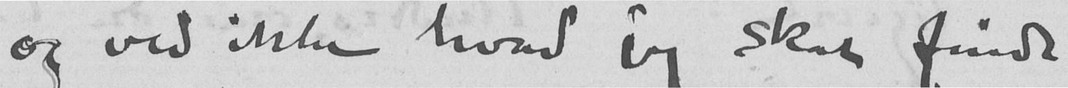og ved ikke hvad jeg skal finde
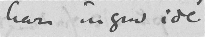har ingen idé
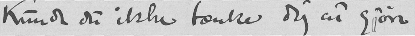Kunde du ikke tænke dig at gjøre
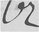Jeg
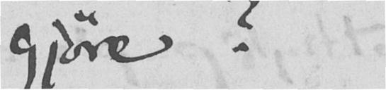gjøre?
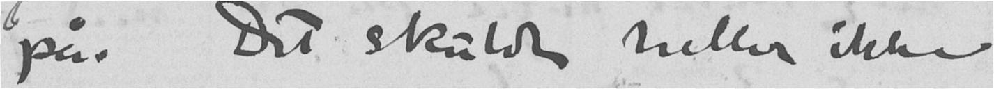på. Det skulde heller ikke
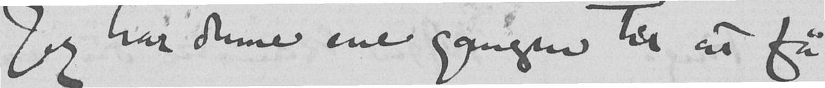Jeg har denne ene gangen til at få
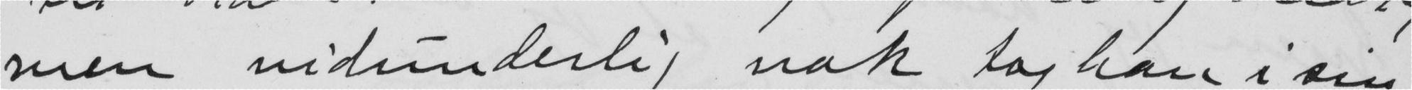men vidunderlig nok tog han i sin
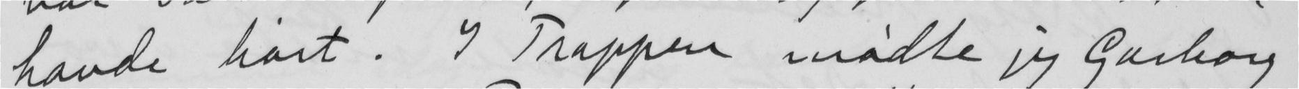havde hørt. I Trappen mødte jeg Garborg
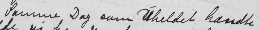Samme Dag som Uheldet hændte
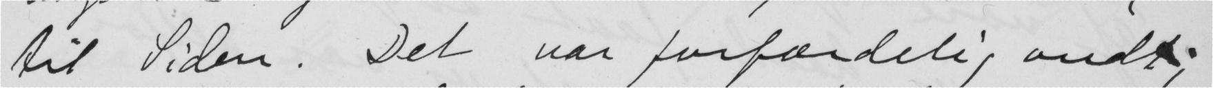til Siden. Det var forfærdelig ondt;
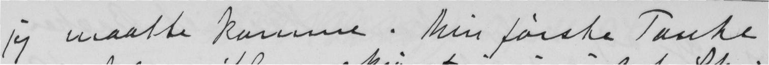jeg maatte komme. Min første Tanke
